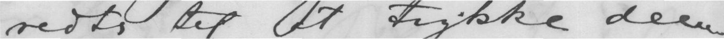redts til at trykke denne
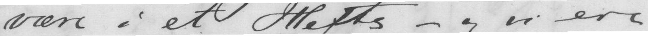være i et Hefts - og vi ere
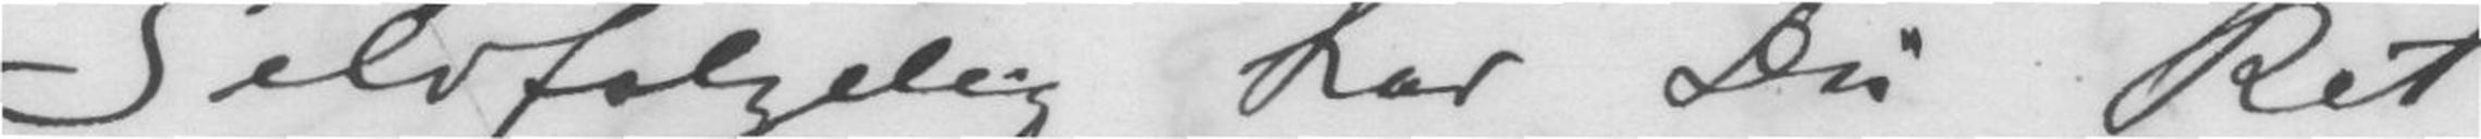Selvfølgelig har Du Ret
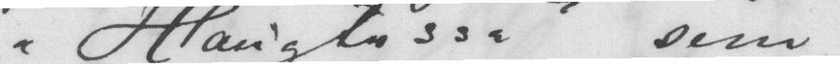"Hangtussa" som
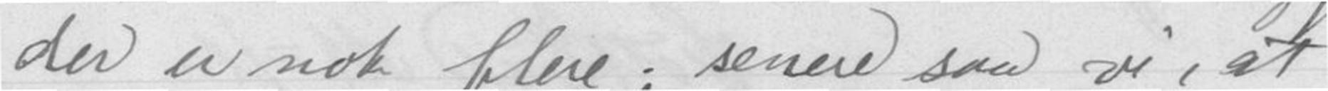der er nok flere; senere saa vi, at
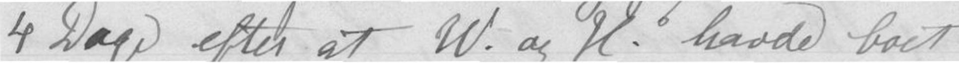4 Dage efter at W. og H. havde boet
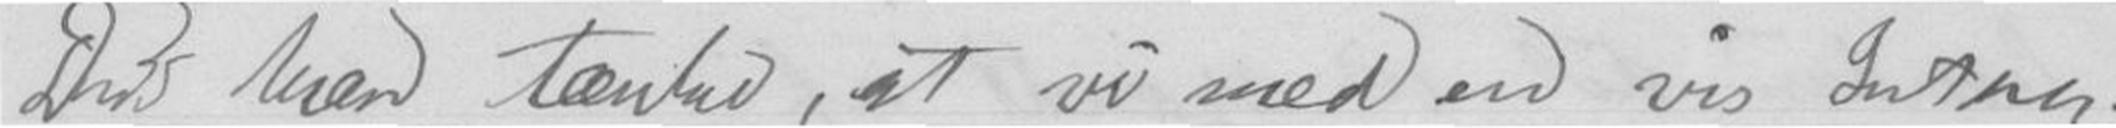Du kan tænke, at vi med en vis Interes-
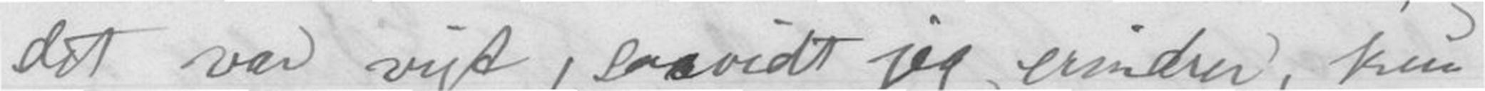det var vist, saavidt jeg erindrer, kun
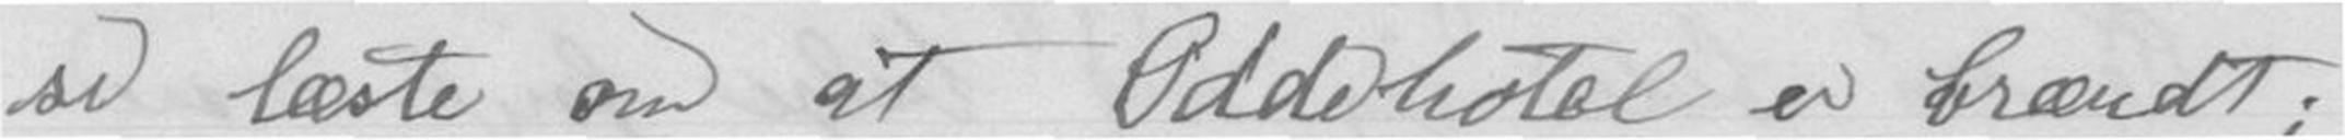se læste om at Oddehotel er brændt;
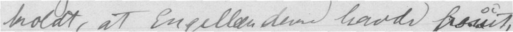koldt, at Engellænderne havde frossit,
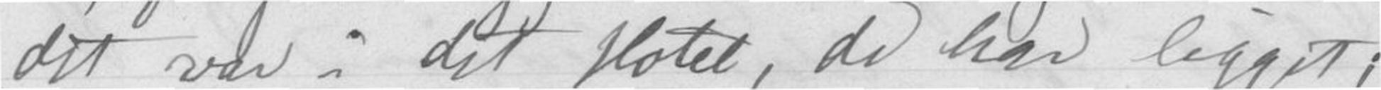det var i det Hotel, de har ligget;
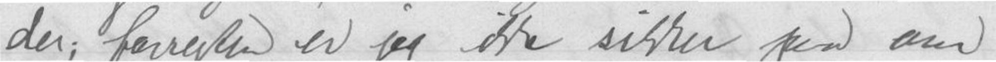der; forresten er jeg ikke sikker paa om
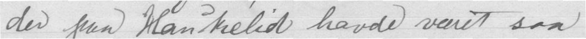der paa Haukelid havde været saa
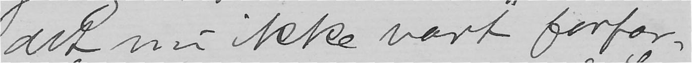det nu ikke vært forfær-
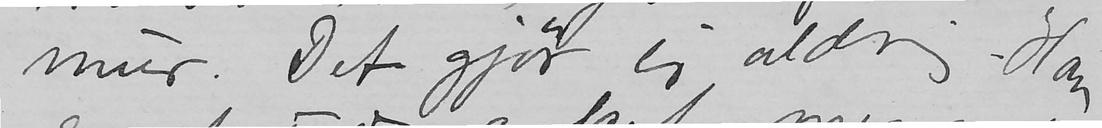mur. Det gjør jeg aldrig. Han
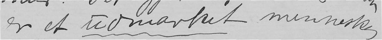er et udmærket menneske
